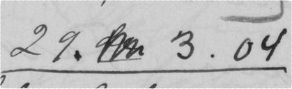29. 3.04
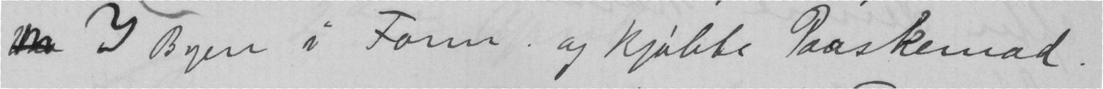I Byen i Form. og kjøbte Paaskemad.
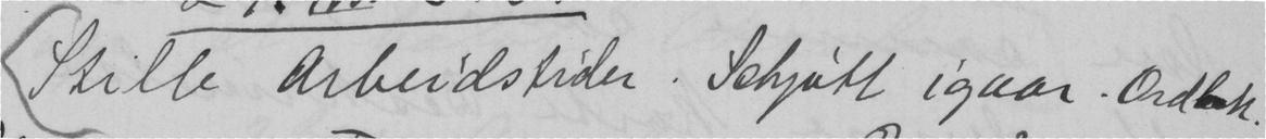Stille arbeidstider. Schjøtt igaar. Ordbok.
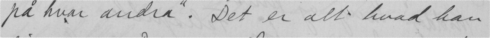på hvar andra". Det er alt hvad han
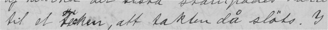til et tecken, att takten då sløts. I
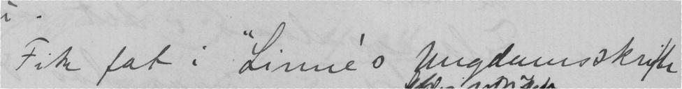Fik fat i "Linnés Ungdomsskrifte
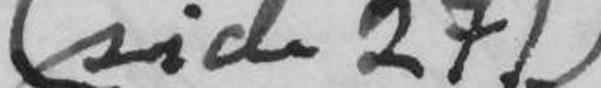(side 271)
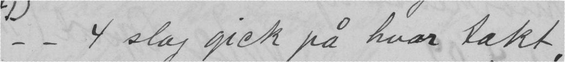- - 4 slag gick på hvar takt,
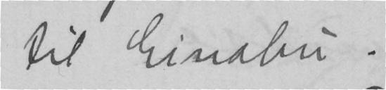til Einabu.
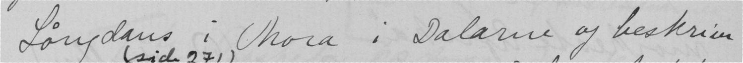Långdans i Mora i Dalarne og beskriver
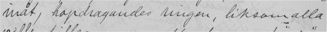inåt, hopdragandes ringen liksom alla
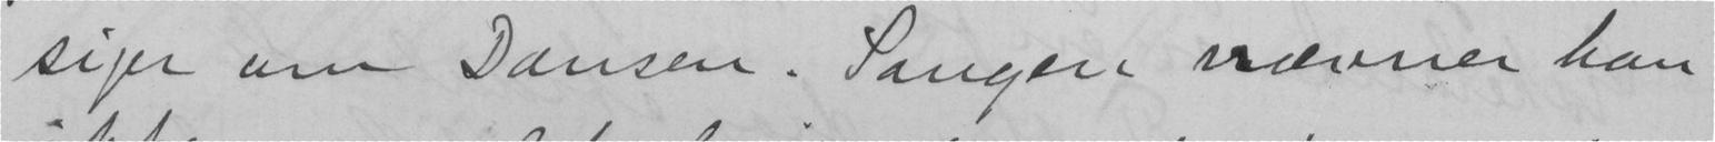siger om Dansen. Sangen nævner han
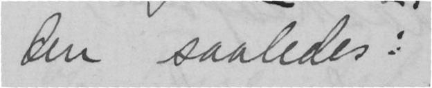den saaledes:
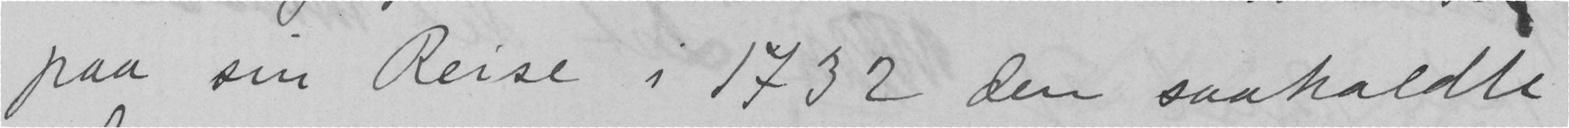paa sin Reise i 1732 den saakaldte
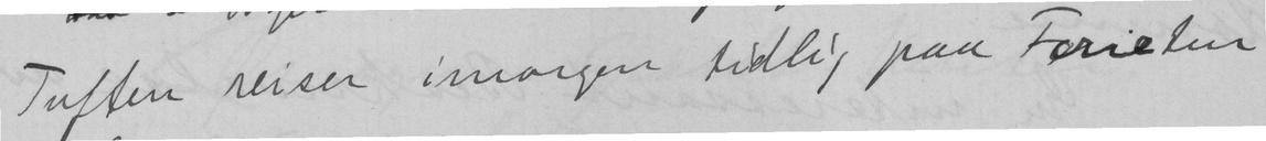Tuften reiser imorgen tidlig paa Ferietun
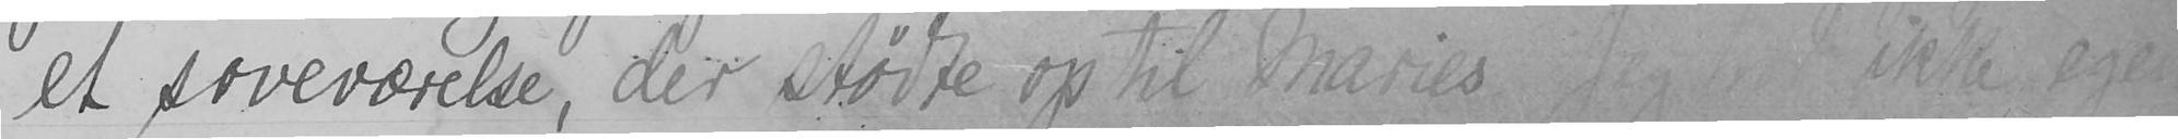et soveværelse, der stødte op til Maries.  Jeg tror ikke egen
